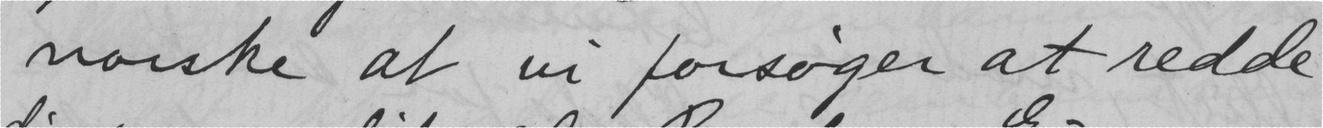norske at vi forsøger at redde
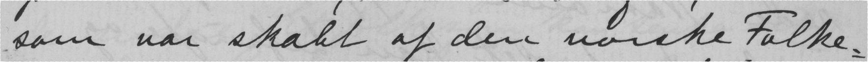som var skabt af den norske Folke-
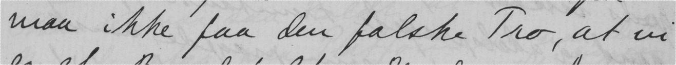maa ikke faa den falske Tro, at vi
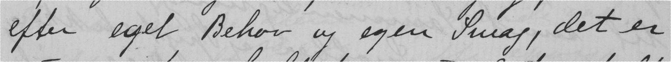efter eget Behov og egen Smag, det er
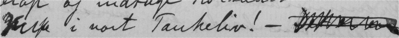virke i vort Tankeliv! -
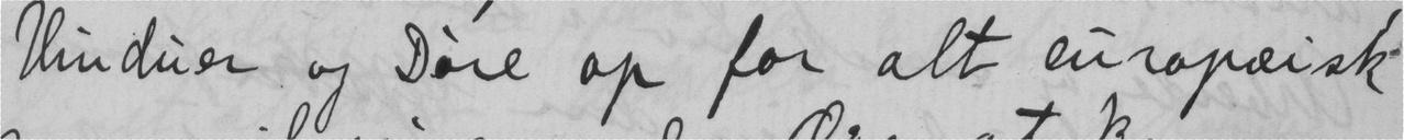Vinduer og Døre op for alt europeisk
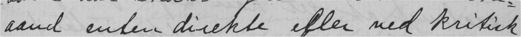aand enten direkte eller ved kritisk
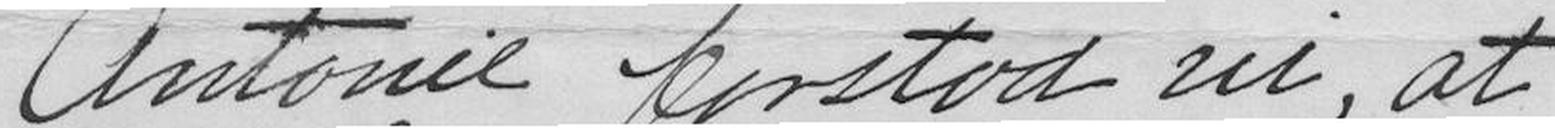Antonie forstod vi, at
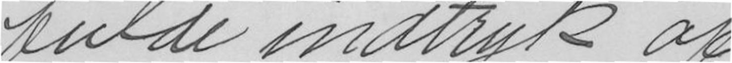fulde indtryk af
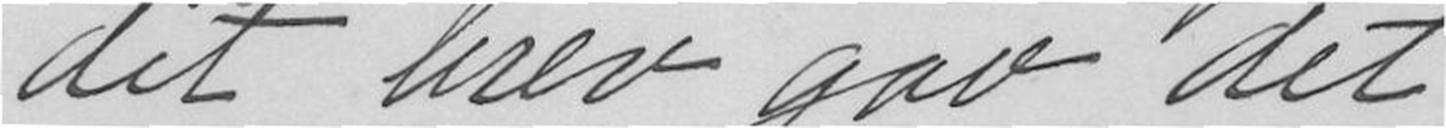dit brev gav det
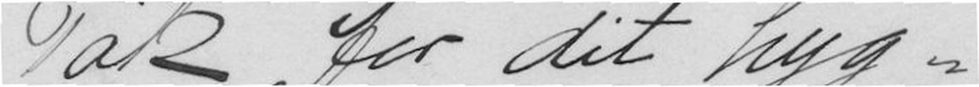Tak for dit hyg-
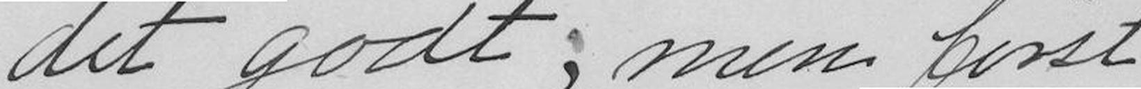det godt; men først
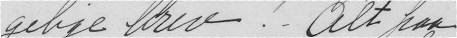gelige brev! Alt paa
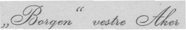Borgen vestre Aker
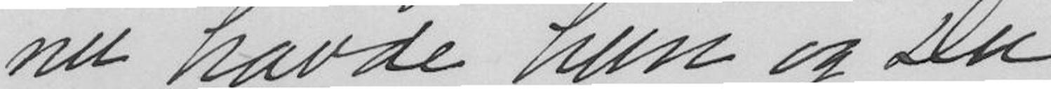nu havde hun og Du
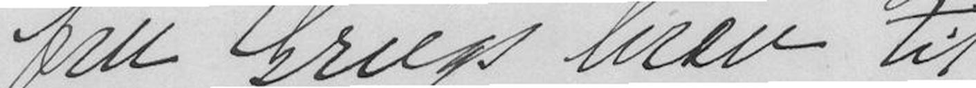fru Griegs brev til
